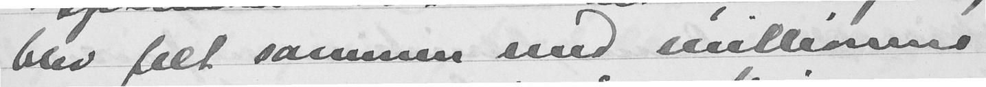blev felt sammen med millioner
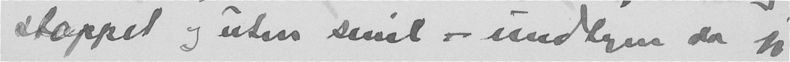stoppet og uten smil - undtagen da jeg
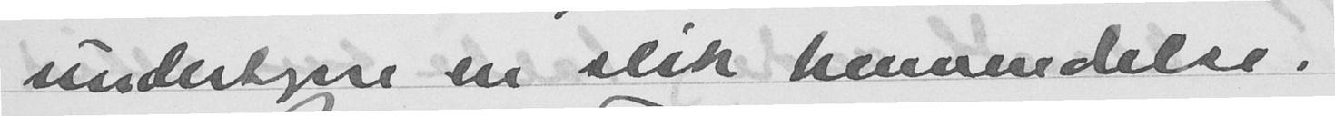undertegne en slik henvendelse.
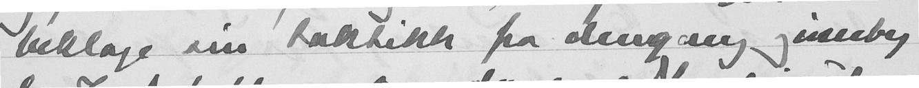beklage sin taktikk fra dengang og tilby
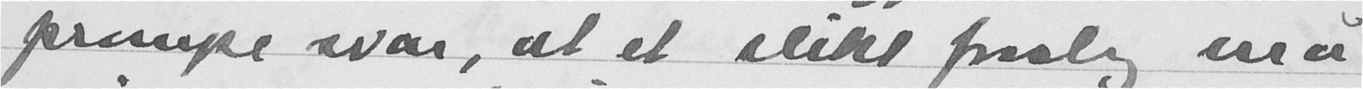propre svar, at et slikt forslag nu
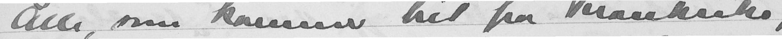Alle, som kommer hit fra Frankrike,
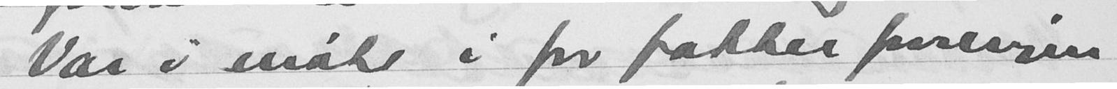Var i møte i forforfatterforeningen
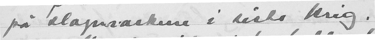på slagmarkene i siste krig. -
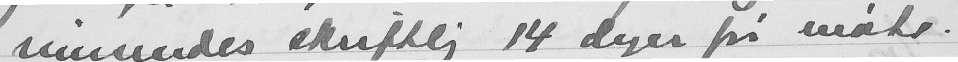innsendes skriftlig 14 dager før møte.
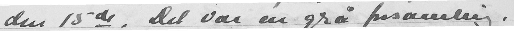den 15de. Det var en bra forsamling.
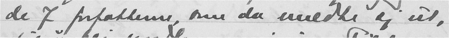de 7 forfatterne som da meldte sig ut,
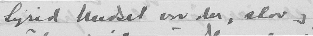Sigrid Undset var der, stor og
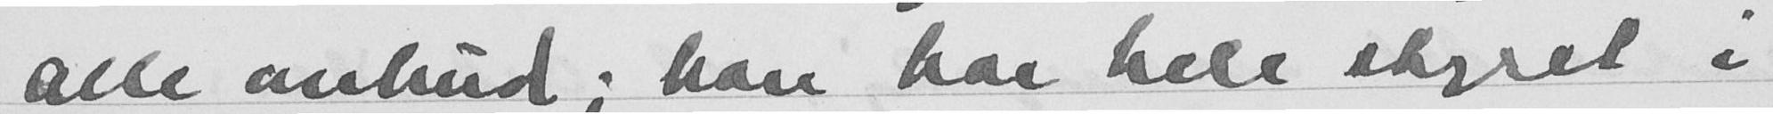alle anbud; han har hele styret i
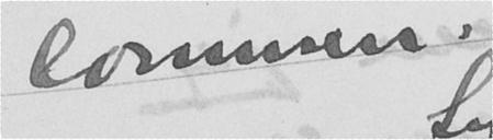lommen.
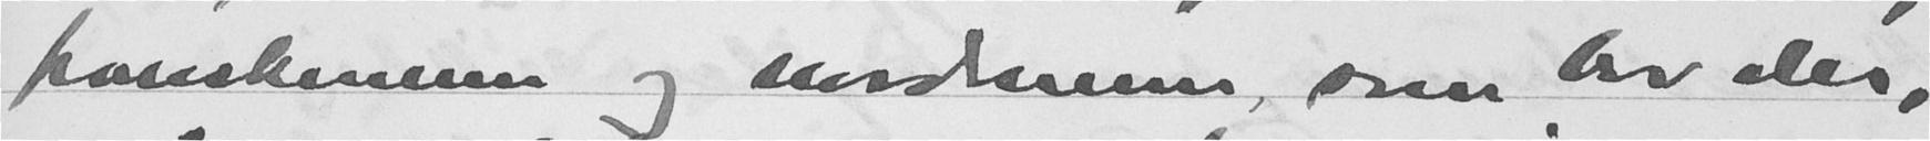franskmenn og nordmenn, som bor der,
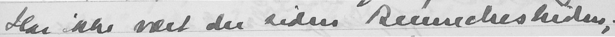Har ikke vært der siden Bennechestriden;
